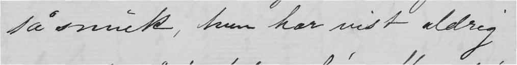så smuk, hun har vist aldrig
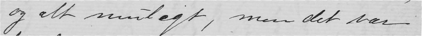og alt muligt, men det var
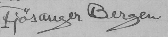Fjøsanger Bergen
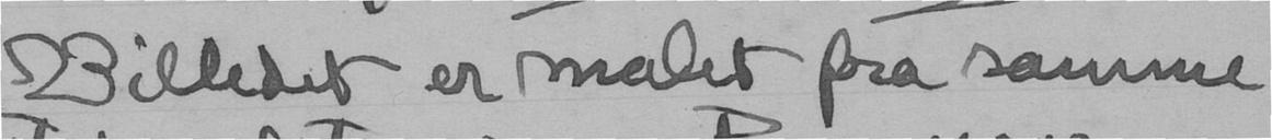Billedet er malet fra samme
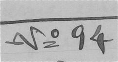No 94
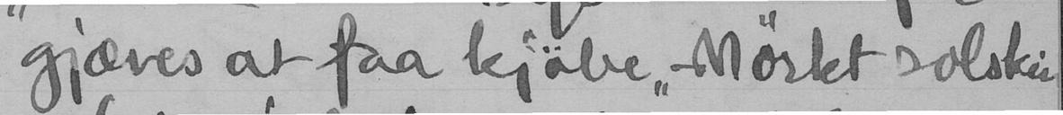gjæves at faa kjøbe "Mørkt solskin"
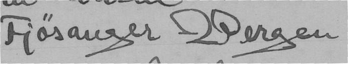Fjøsanger Bergen
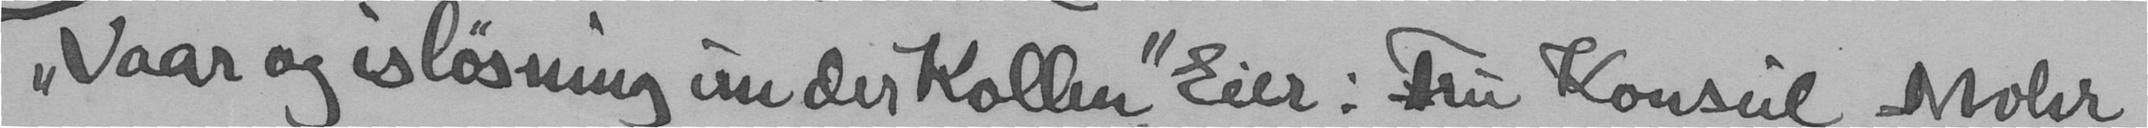"Vaar og isløsning under Kollen" Eier: Fru Konsul Mohr
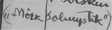("Mørk solmystik")
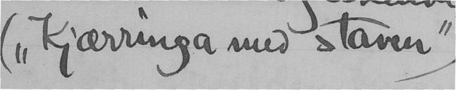("Kjærringa med staven")
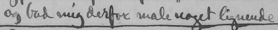og bad mig derfor male noget lignende
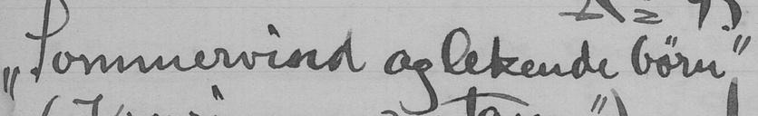"Sommervind og lekende børn"
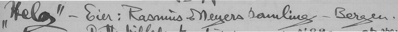"Helg" - Eier: Rasmus Meyers samling - Bergen.
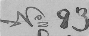No 93
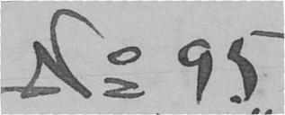No 95
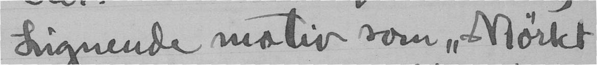Lignende motiv som "Mørkt
